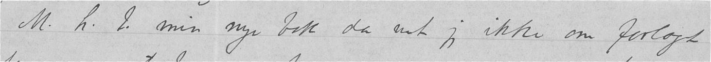M.h.t. min nye bok da vet jeg ikke om forlaget
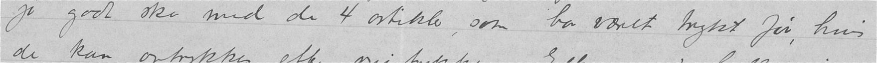jo godt ske med de 4 artikler, som har været trykt før, hvis
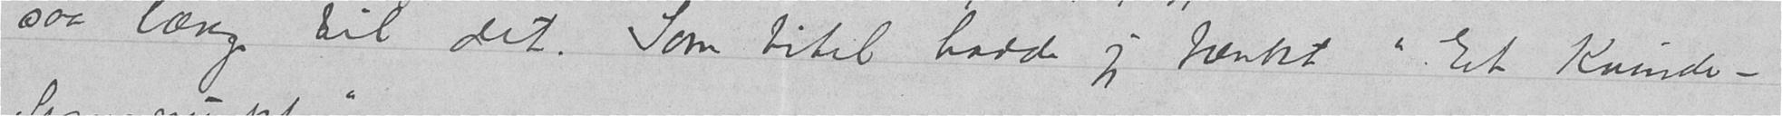saa længe til det. Som titel hadde jeg tænkt «Et Kvinde –
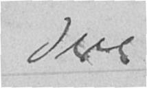Deres
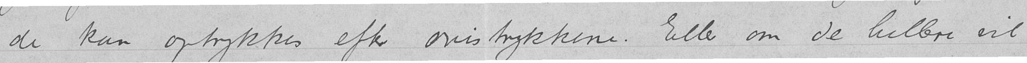de kan optrykkes efter avistrykkene. Eller om de hellere vil
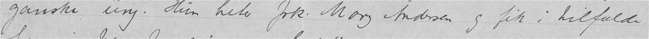ganske, ung. Hun heter frk. Mary Andersen og fik i tilfælde
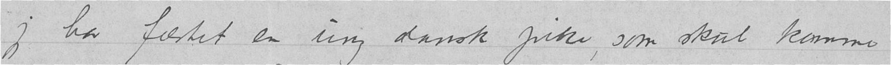jeg har fæstet en ung dansk pike, som skal komme
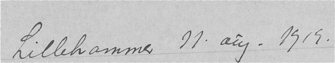Lillehammer 11. aug. 1919.
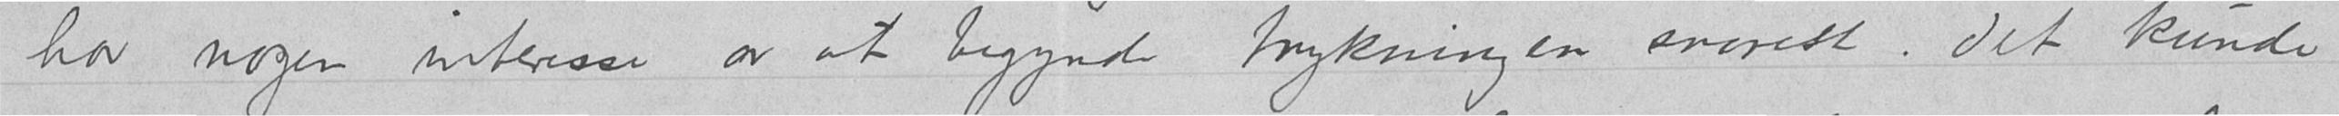har nogen interesse av at begynde trykningen snarest. Det kunde
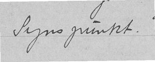Synspunkt.»
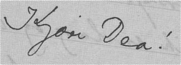Kjære Dea!
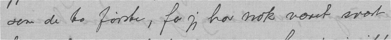som de to første, for jeg har nok været svært
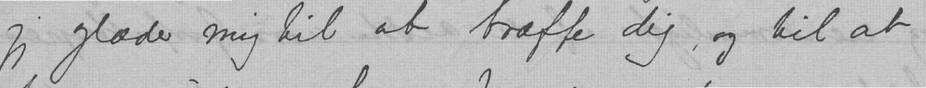jeg glæder mig til at træffe dig, og til at
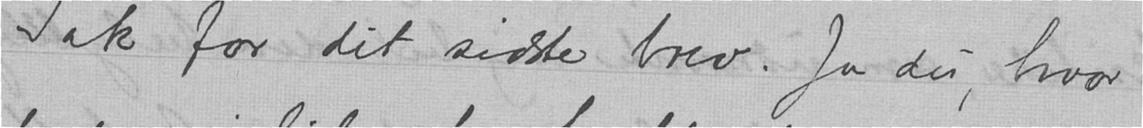Tak for dit sidste brev. Ja du, hvor
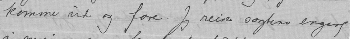komme ud og fare. Jeg reiser sagtens engang
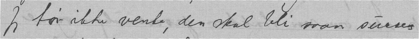Jeg tør ikke vente, den skal bli saan success
